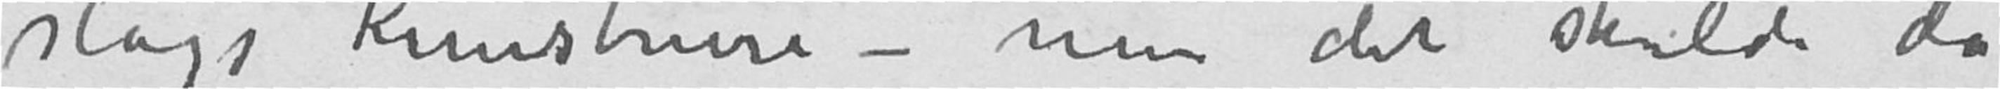slags Kunstnere – men det skulde da
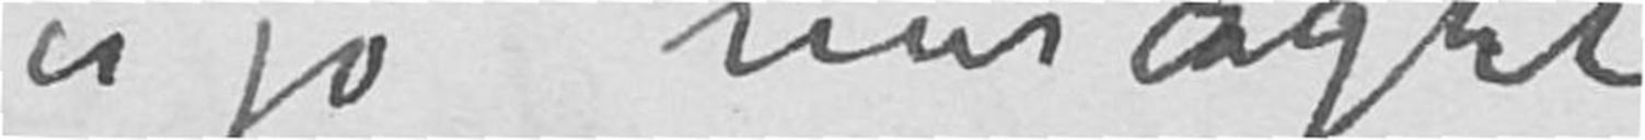er jo mer agtet
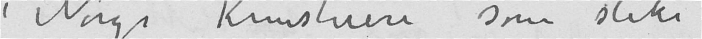i Norge Kunstnere som slike –
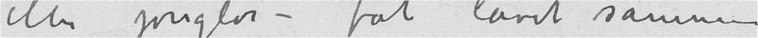eller jonglør» – fåt lavet sammen
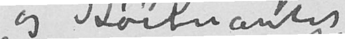og Løitnanter
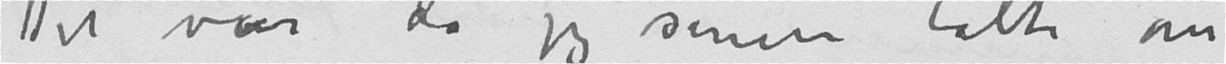Det var da jeg senere talte om
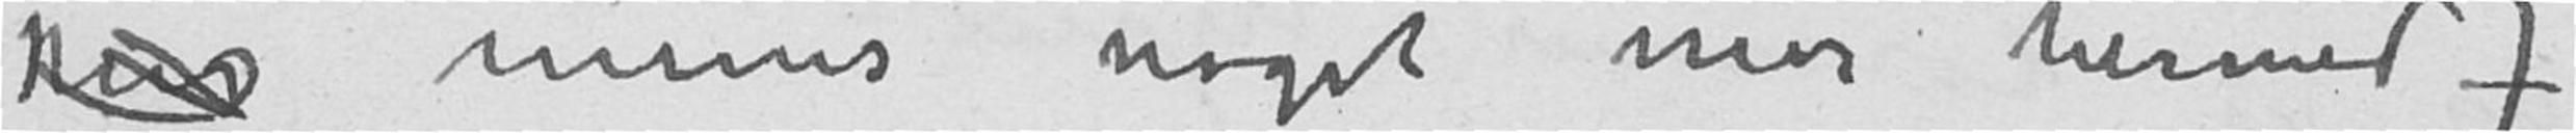menes noget mer herved –)
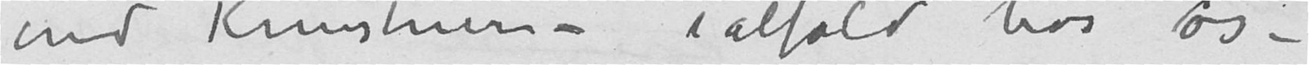end Kunstnere – ialfald hos os –
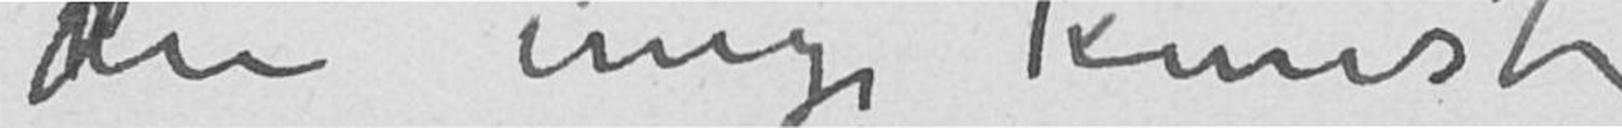den unge Kunst
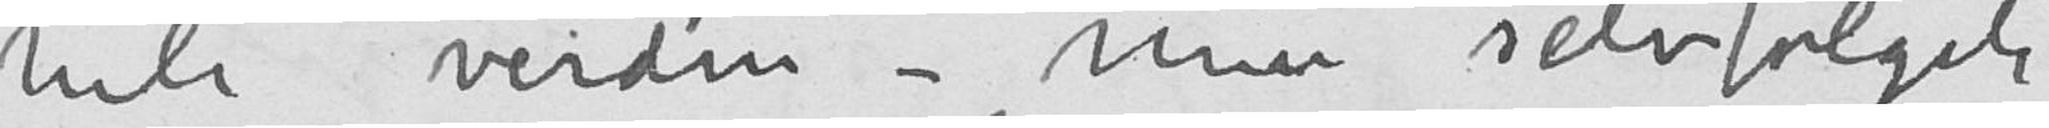hele verden – Men selvfølgeli
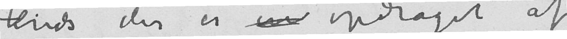–kreds der er en opdraget af
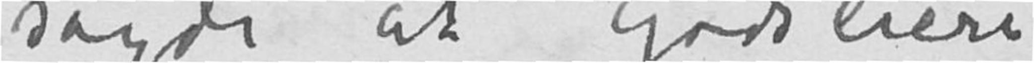sagde at Godseiere
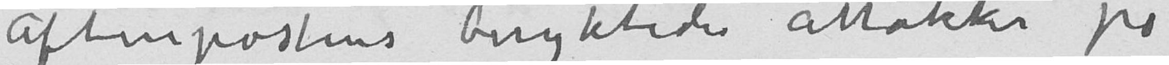Aftenpostens beryktede attakker på
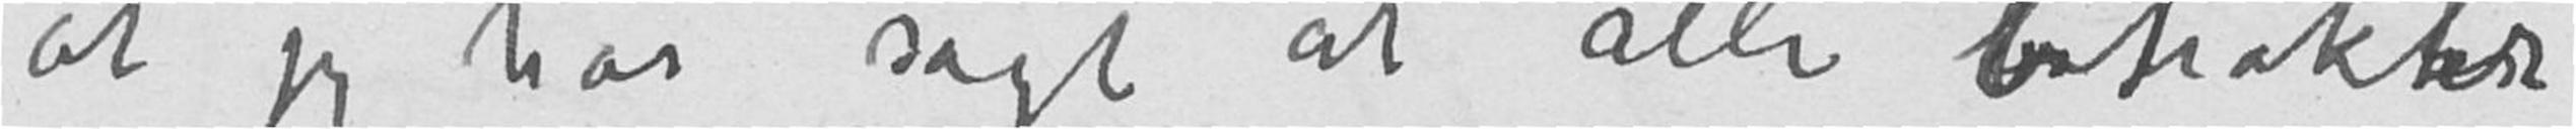at jeg har sagt at alle betrakter
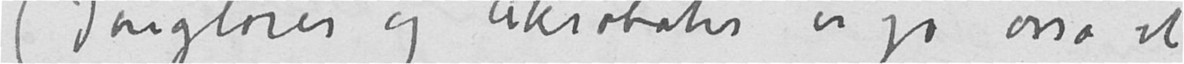(Jonglører og Akrobater er jo osså et
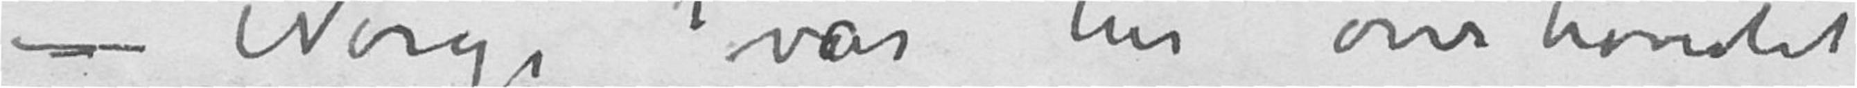– Norge var her overhovedet
